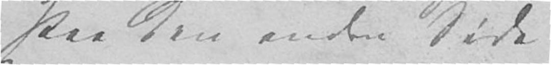Paa den anden Side
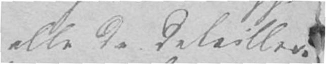alle de detaillere-
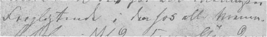forpligtende i den hos alle Menne-
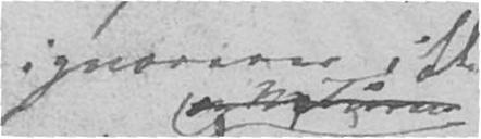ignorerer ikke
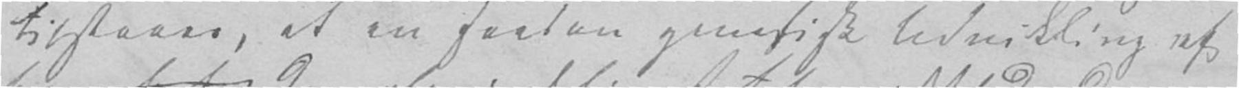tilstaaes, at en saadan genetisk Udvikling af
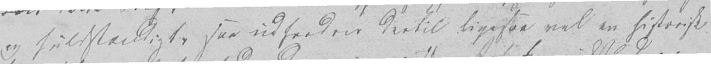og fuldstændigt, saa udfordres dertil ligesaa vel en historisk
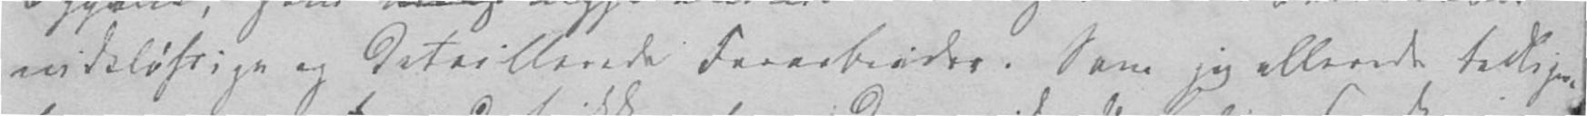vidtløftige og detaillerede Forarbeider. Som jeg allerede tidligere
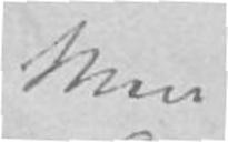Men
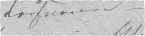Forsvarere.
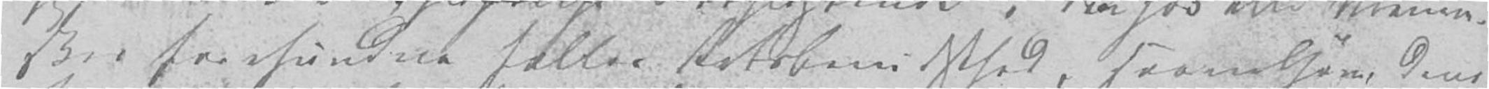sker forefundne fælles Retsbevidsthed, saavelsom dens
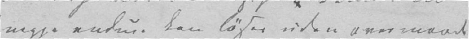neppe endnu kan løses uden overmaade
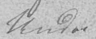Under
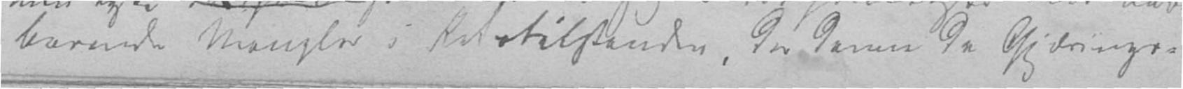barende Mangler i Retstilstanden, der danne de Gjærings-
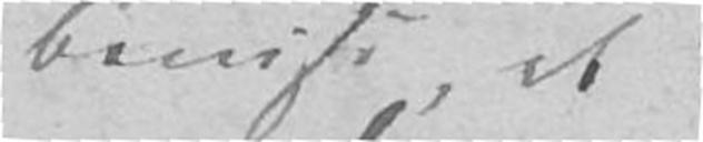bevise, at
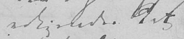erkjender det
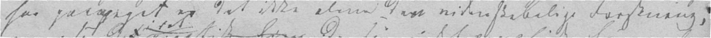har paapeget er det ikke alene den videnskabelige Forskning,
